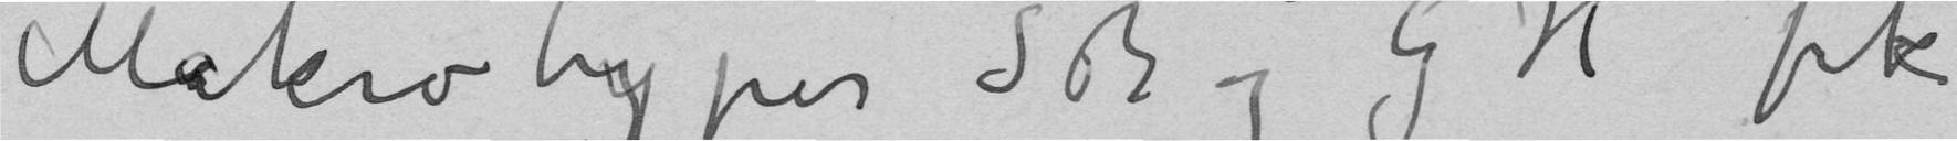makrotyper S B og G H fik
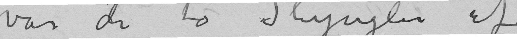var de to Slyngler af
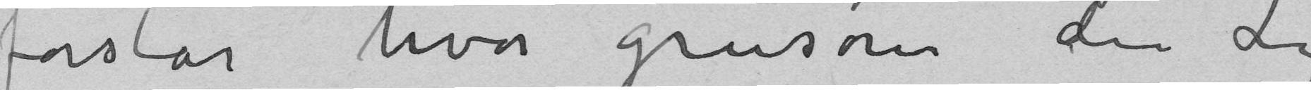forstår hvor grusom den Leg
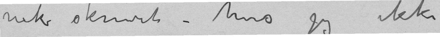nok skrevet – hvis jeg ikke
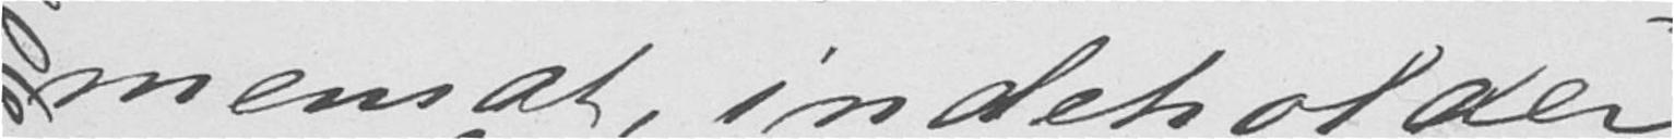mensat, indeholder
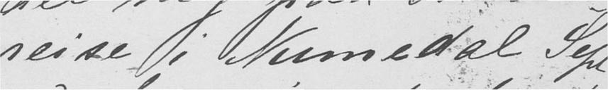reise i Numedal Sept
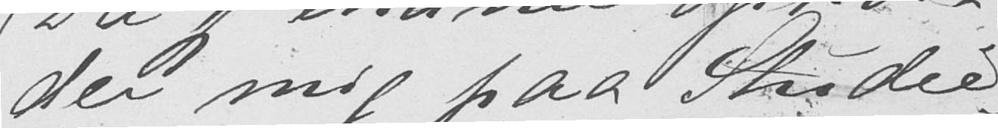der mig paa Studie-
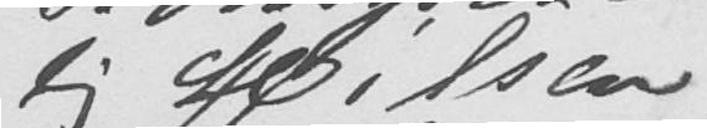lig Hilsen
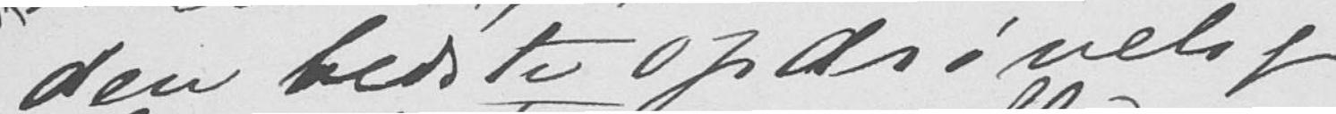den bedste opdrivelige
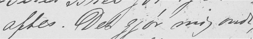aftes. Det gjør mig ondt,
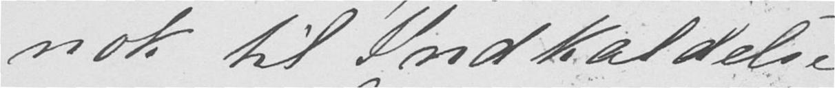nok til Indkaldelse
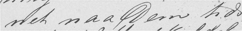net naa Dem tids-
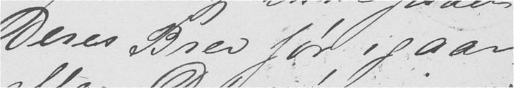Deres Brev før igaar-
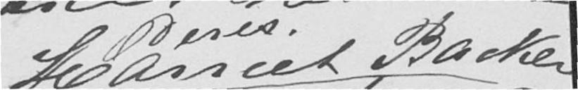Harriet Backer
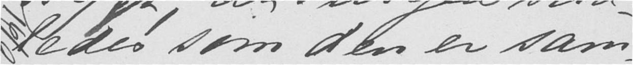ledes som den er sam-
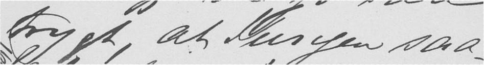trygt, at Juryen saa-
